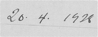20.4.1928
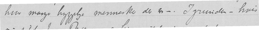hvor mange hyggelige mennesker der er –. Igrunden – hvis
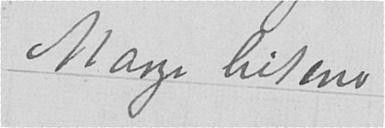Mange hilsener
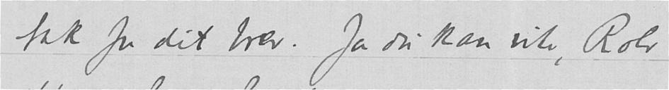tak for dit brev. Ja du kan vite, Rolv
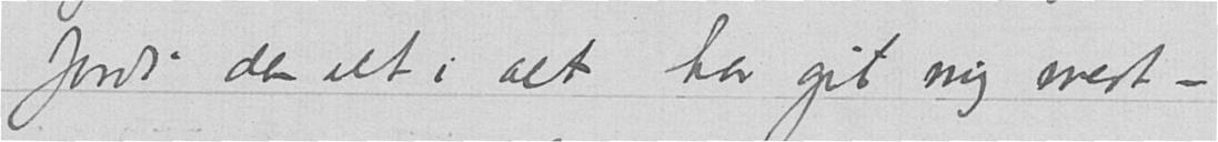Fordi den alt i alt har git mig mest –.
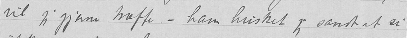vil jeg gjerne træffe – ham husket jeg sandt at si
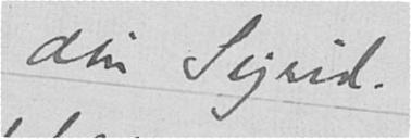din Sigrid.
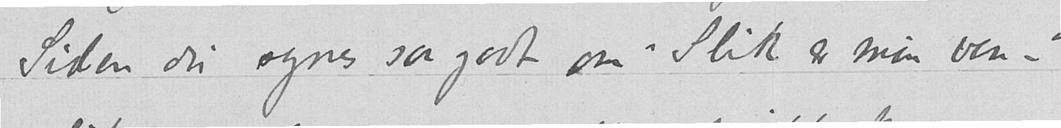Siden du synes saa godt om "Slik er min ven -"
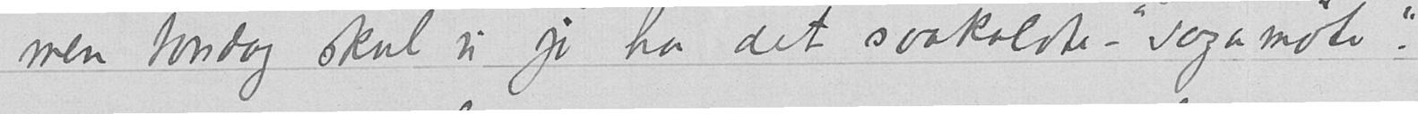men torsdag skal vi jo ha det saakaldte – "sagamøte".
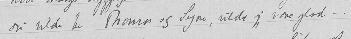du vilde be Thomas og Signe, vilde jeg være glad -.
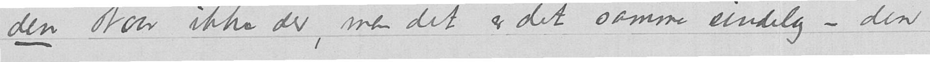den staar ikke der, men det er det samme sindelag – den
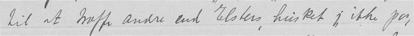til at træffe andre end Elsters, husket jeg ikke paa,
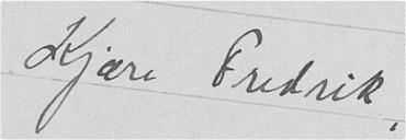Kjære Fredrik,
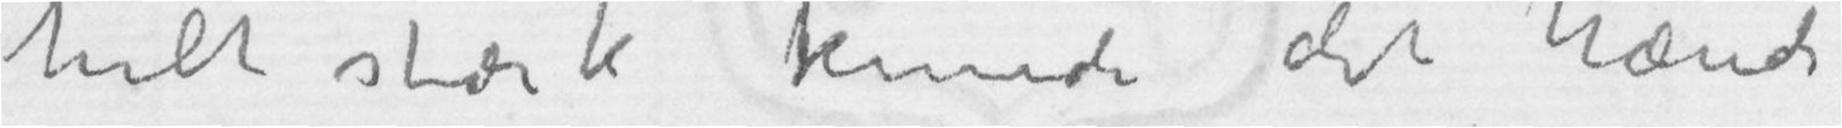helt stærk kunde det hænde
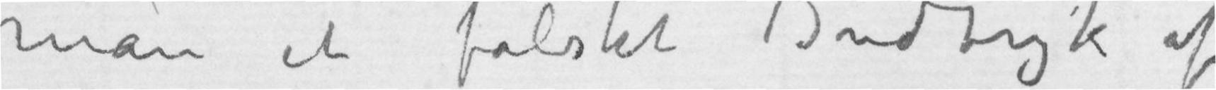man et falskt Indtryk af
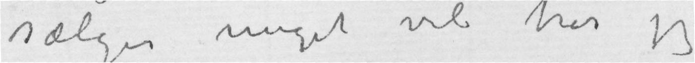sælger meget vil tror jeg
